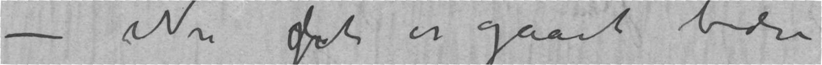– Nu det er gaaet bedre
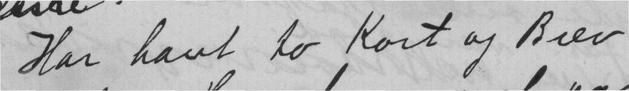Har havt to Kort og Brev
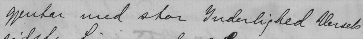gjentar med stor Inderlighed Versets
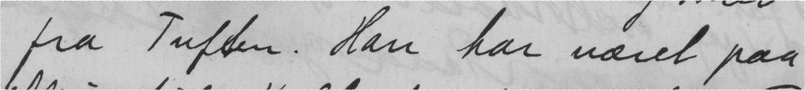fra Tuften. Han har været paa
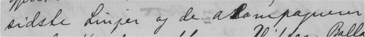sidste Linjer og de akompagnerer
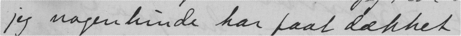jeg nogenlunde har faat dækket
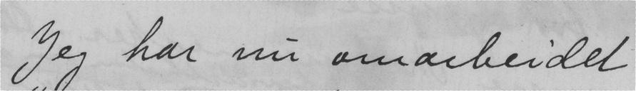Jeg har nu omarbeidet
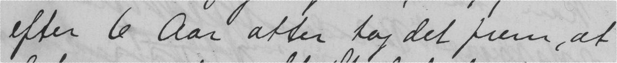efter 6 Aar atter tog det frem, at
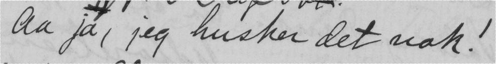Aa ja, jeg husker det nok!
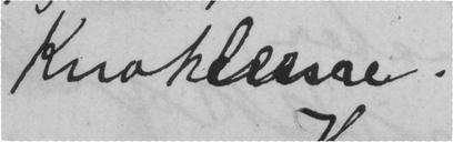Knoklerne.
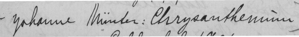Johanne Münter: Chrysanthemum
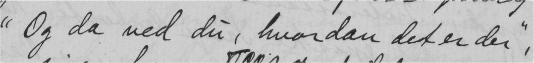"Og da ved du, hvordan det er der",
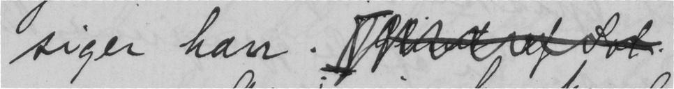siger han.
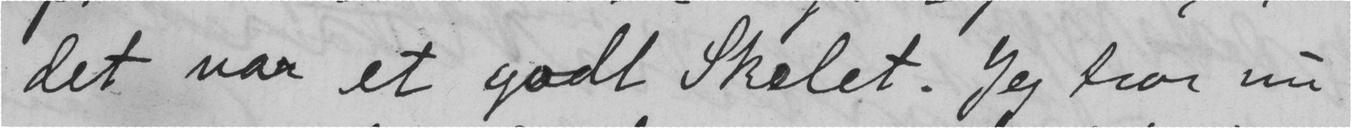det var et godt Skelet. Jeg tror nu
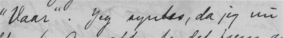"Vaar". Jeg syntes, da jeg nu
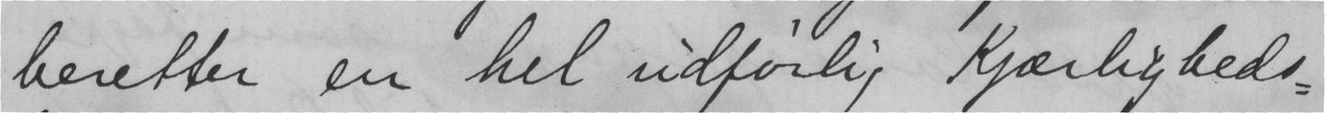beretter en hel udførlig Kjærligheds-
